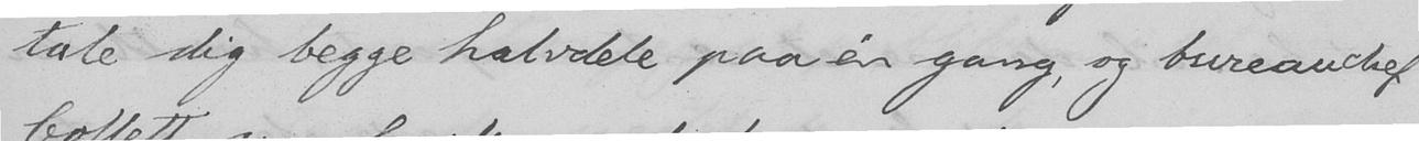tale dig begge halvdele paa én gang, og bureauchef
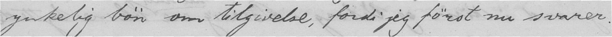ynkelig bøn om tilgivelse, fordi jeg først nu svarer.
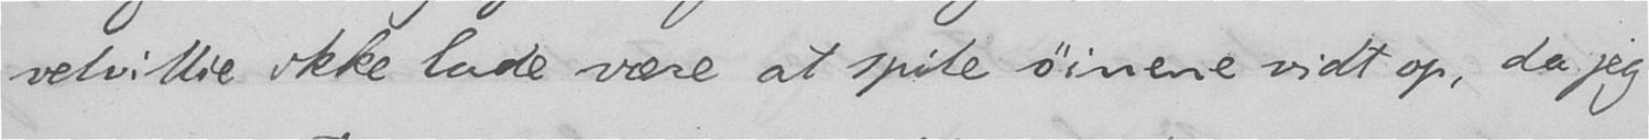velvillie ikke lade være at spile øinene vidt op, da jeg
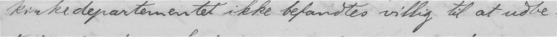kirkedepartementet ikke befandtes villig til at udbe-
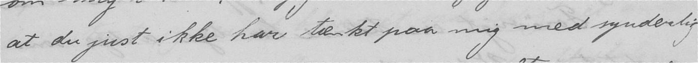at du just ikke har tænkt paa mig med synderlig
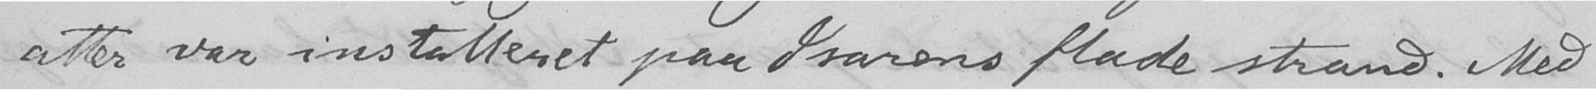atter var installeret paa Isarens flade strand. Med
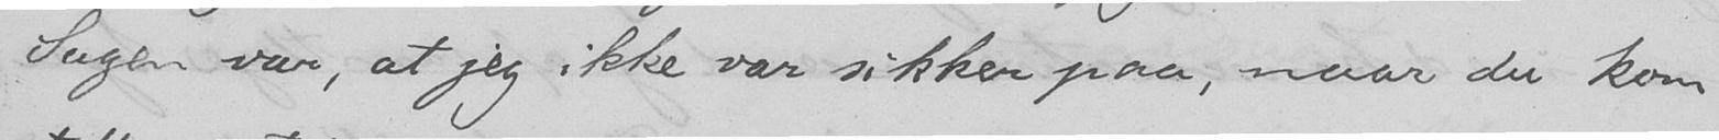Sagen var, at jeg ikke var sikker paa, naar du kom
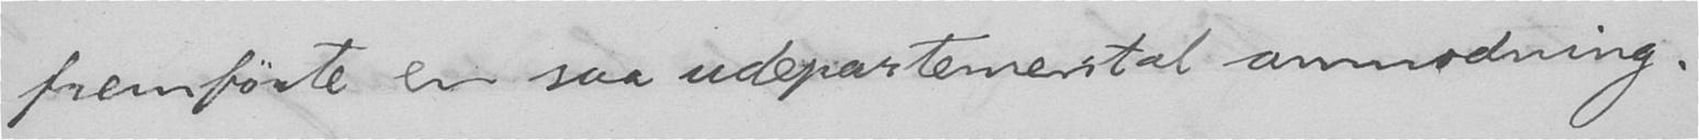fremførte en saa udepartemental anmodning.
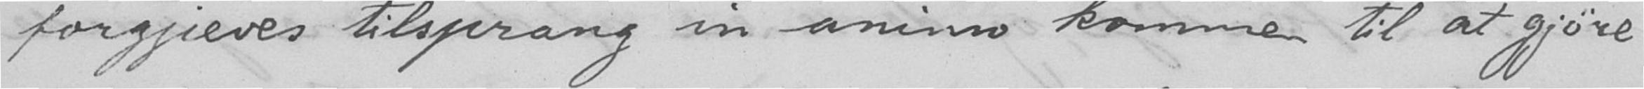forgjæves tilsprang in anima kommen til at gjøre
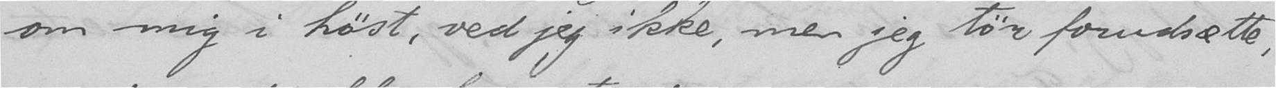om mig i høst, ved jeg ikke, men jeg tør forudsætte,
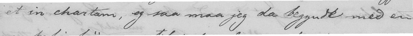et in chartam, og saa maa jeg da begynde med en
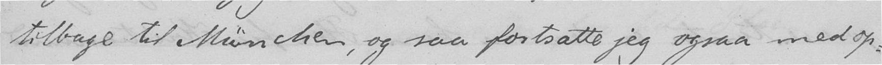tilbage til München, og saa fortsatte jeg ogsaa med op-
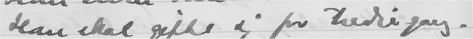Han skal gifte sig for tredje gang.
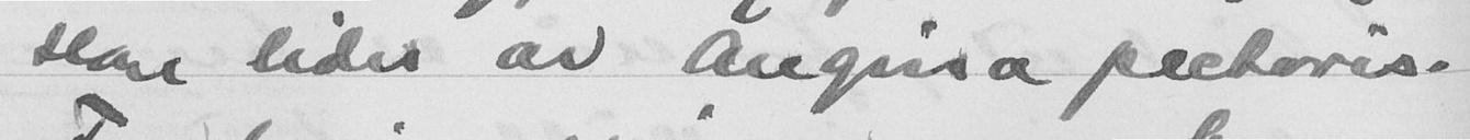Han lider av Augenia paeloris.
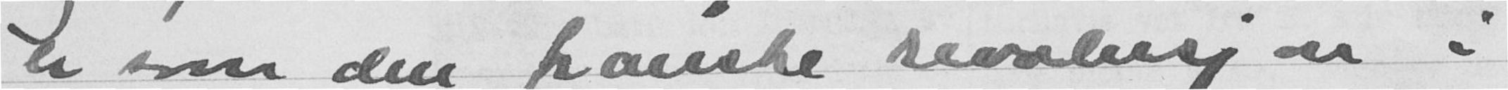er som den franske revolusjon i
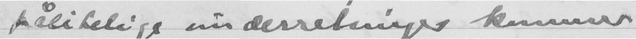pålitelige underretninger kommer
